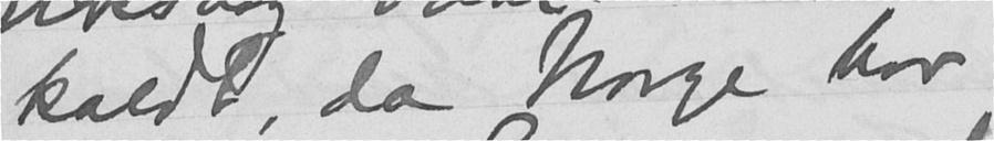kaldt, da Norge har
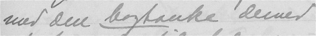med den bagtanke dermed
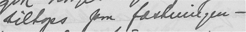tiltops paa fæstningen -
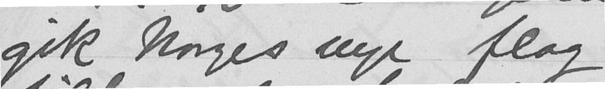gik Norges nye flag
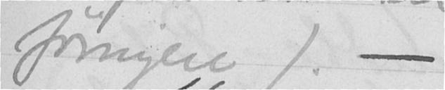føringen). -
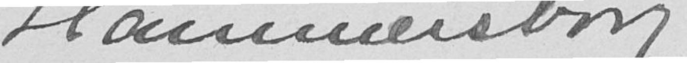Hammersborg
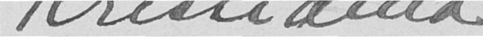Kristiania
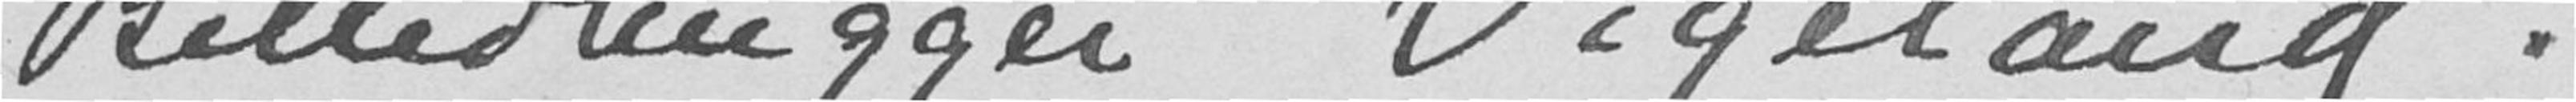Billedhugger Vigeland.
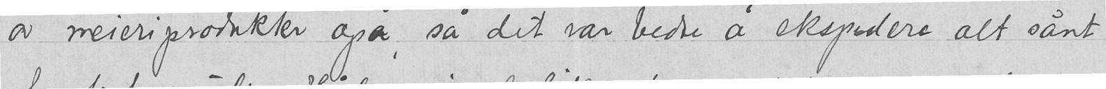av meieriprodukter også, så det var bedre å ekspedere alt sånt
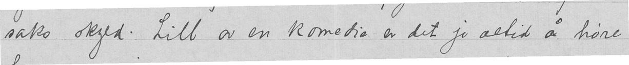saks skyld. Litt av en komedie er det jo altid å høre
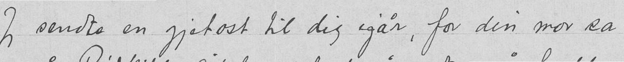Jeg sendte en gjetost til dig igår, for din mor sa
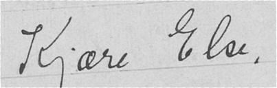Kjære Else,
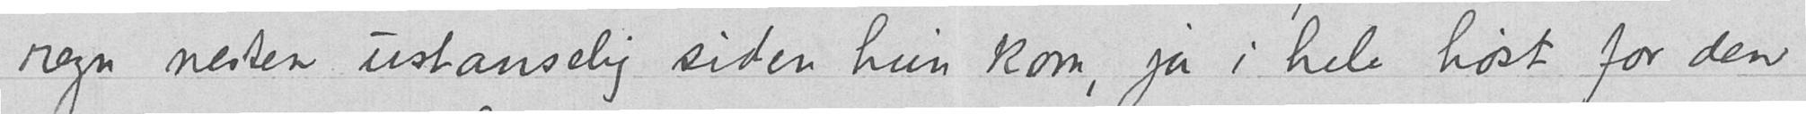regn nesten ustanselig siden hun kom, ja i hele høst for den
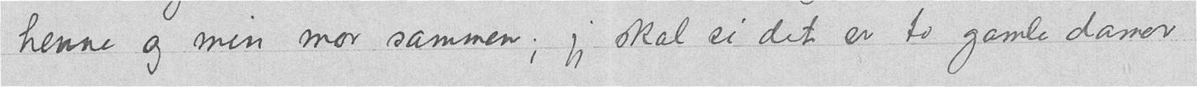henne og min mor sammen; jeg skal si det er to gamle damer
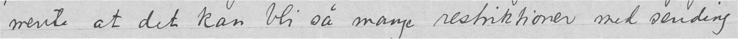mente at det kan bli så mange restriktioner med sending
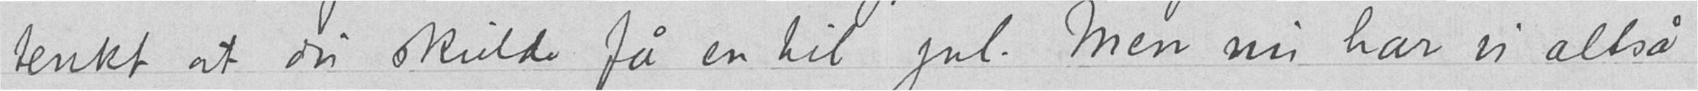tenkt at du skulde få en til jul. Men nu har vi altså
